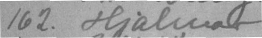162. Hjalmar
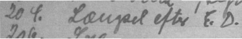204. Længsel efter E.D.
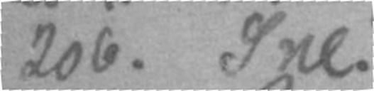206. Sne.
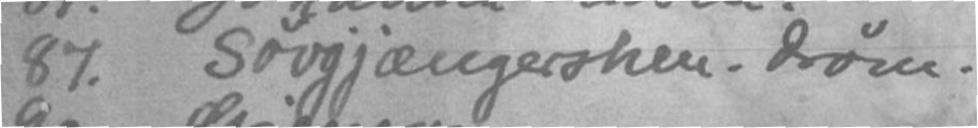87. "Søvngjængersken - drøm.
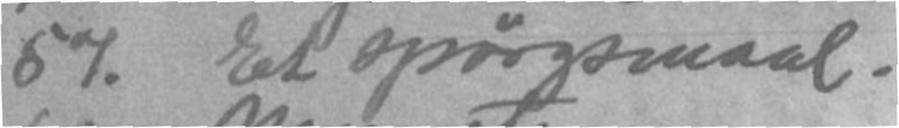57. Et spørgsmaal.
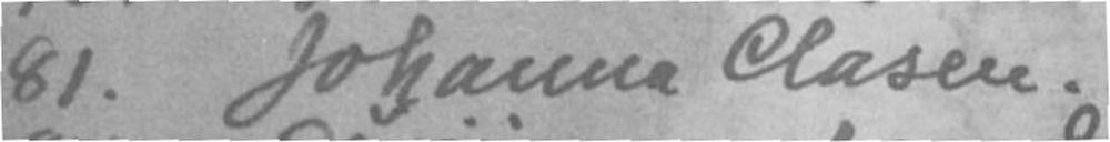81. Johanna Clasen.
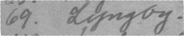69. Lyngby.
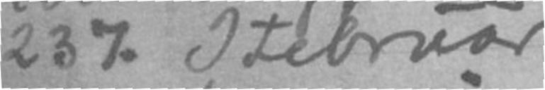237. I februar
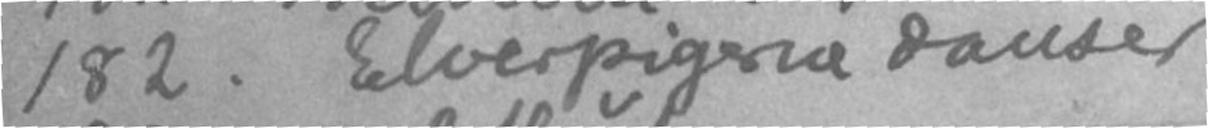182. Elverpigerne danser
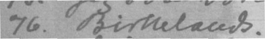76. Birkelands.
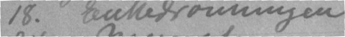18. Enkedronningen
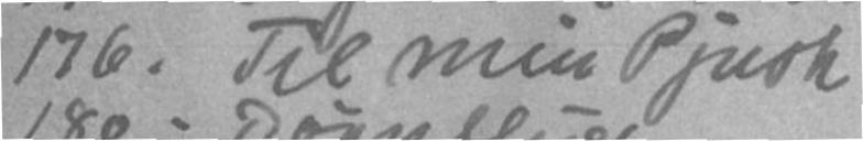176. Til min Pjusk
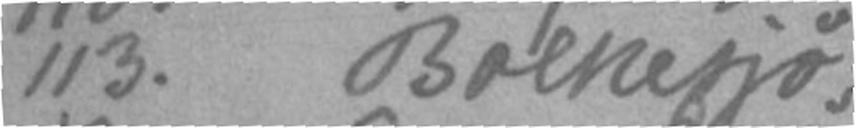113. Bolkesjø.
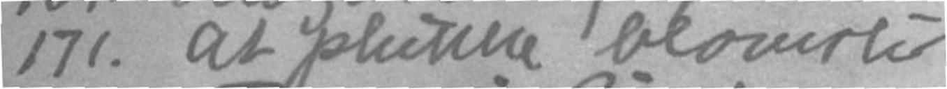171. at plukke blomster
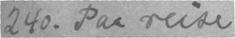240. Paa reise
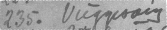235. Vuggesang
